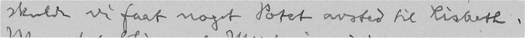skulde vi faat noget Potet avsted til Lisbeth.
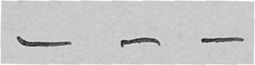- - -
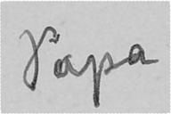Papa
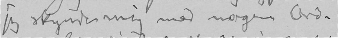jeg skynde mig med nogen Ord.
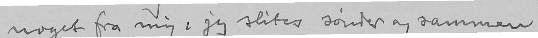noget fra mig, jeg slites sønder og sammen
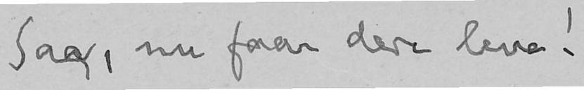Saa, nu faar dere leve!
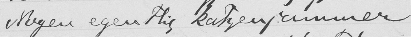Nogen egentlig katzenjammer
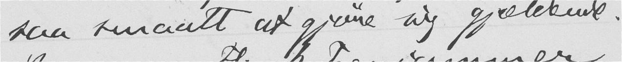saa smaatt at gjøre sig gjældende.
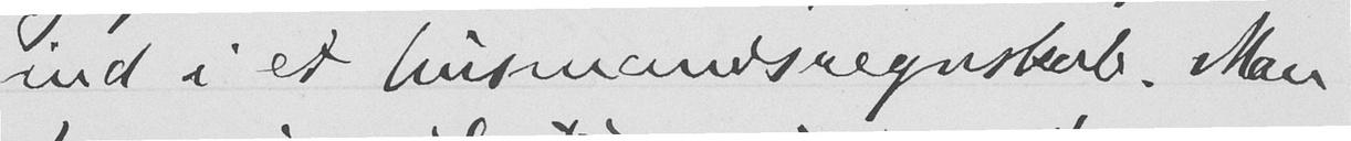ind i et husmandsregnskab. Man
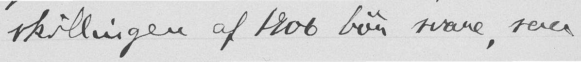skillingen af 1906 bør svare, saa
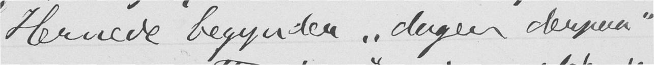Hernede begynder "dagen derpaa"
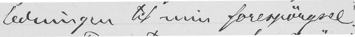ledningen til min forespørgsel.
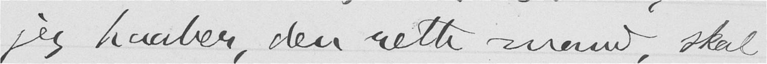jeg haaber, den rette mand, skal
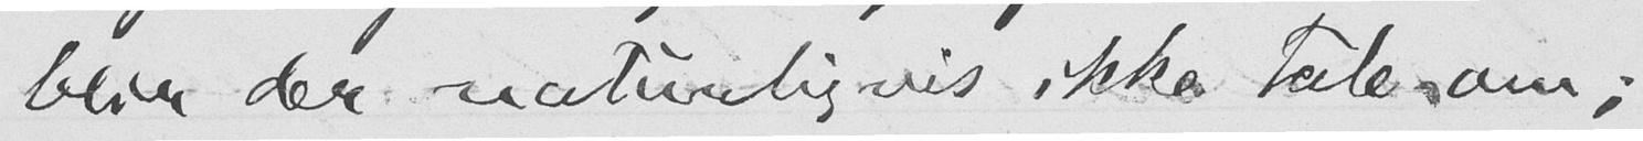blir der naturligvis ikke tale om;
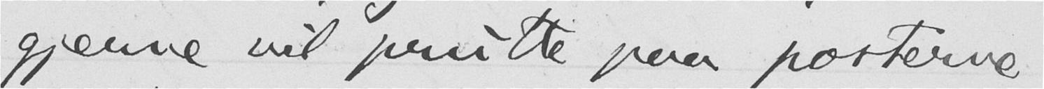gjerne vil prutte paa posterne
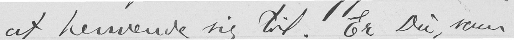at henvende sig til. Er Du, som
